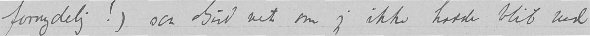fornydelig!) saa Gud vet om jeg ikke hadde blit ved
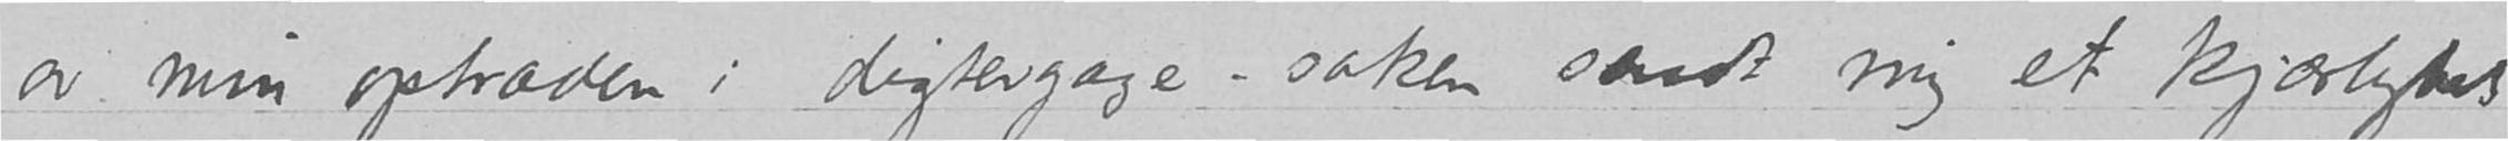av min optræden i digtergage-saken seændt mig et kjærlighets
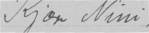Kjære Nini
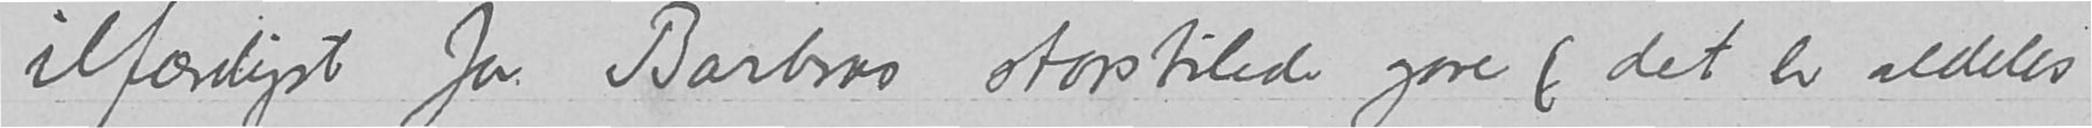ilfærdigst for Barbras storstilede gave (det er aldeles
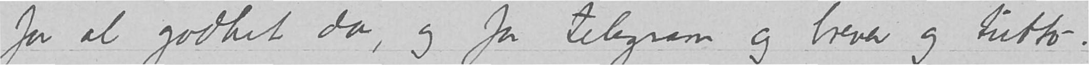for al godhet da, og for telegram og brever og tutto -.
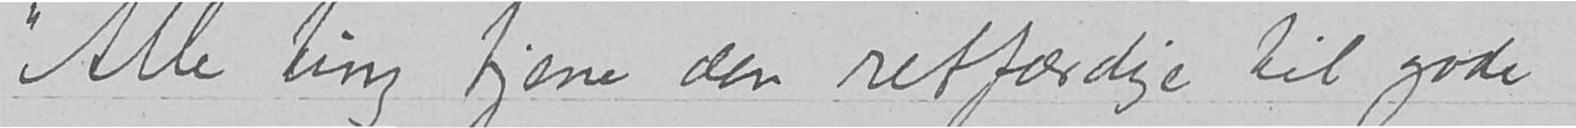«Alle ting tjener den retfærdige til gode»
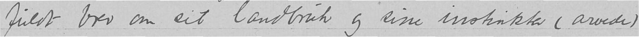fuldt brev om sit landbruk og sine instinkter (arvede)
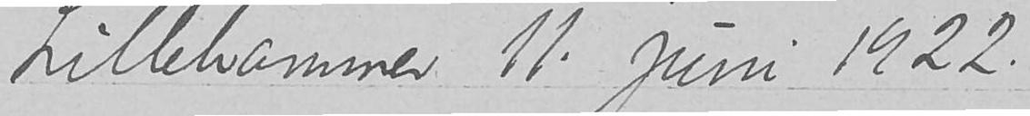Lillehammer 11.juni 1922.
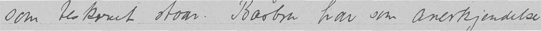som beskrevet staar.  Barbra har som anerkjendelse
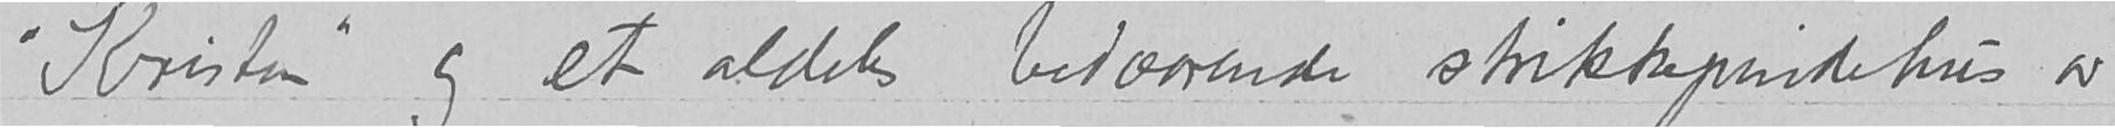«Kristin» og et aldeles bedaarende strikkepindehus av
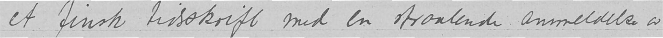et finsk tidsskrift med en straalende anmeldelse av
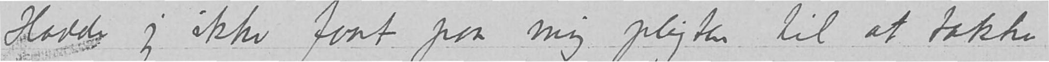Hadde jeg ikke faat paa mig pligtenr til at takke
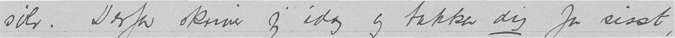sølv. Derfor skriver jeg i dag og takker deg for sisst,
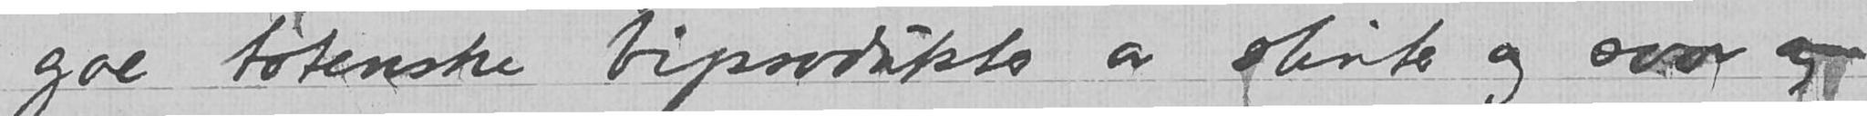goe totenske biprodukter av slintrer og svor og
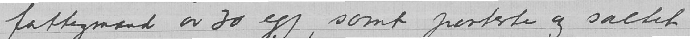fattigmand av 30 egg, samt parterte og saltet
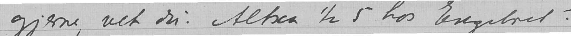gjerne, vet du. Altsaa ½ 5 hos Engebret?
